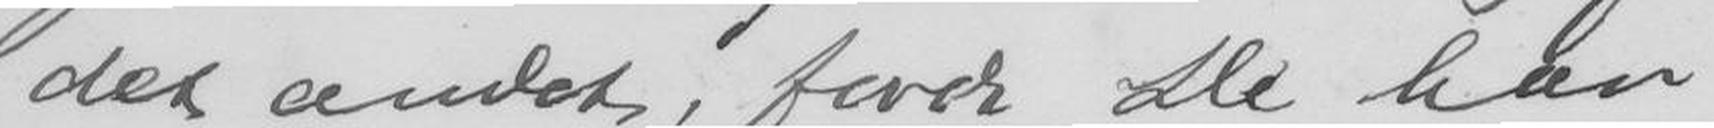det andet, fordi De har
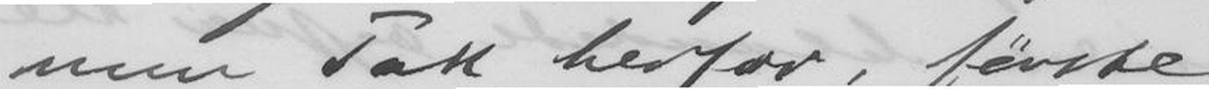min Tak herfor, første
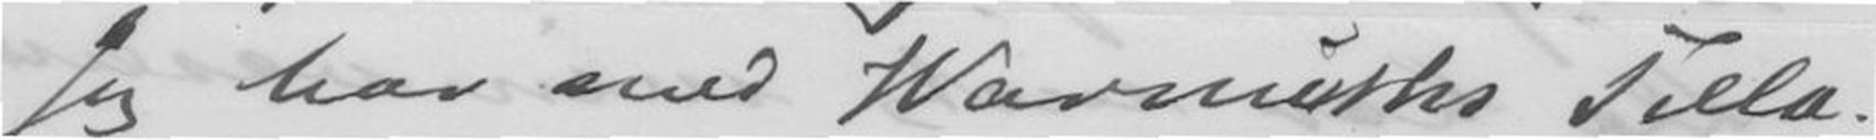Jeg har med Warmuths Tilla-
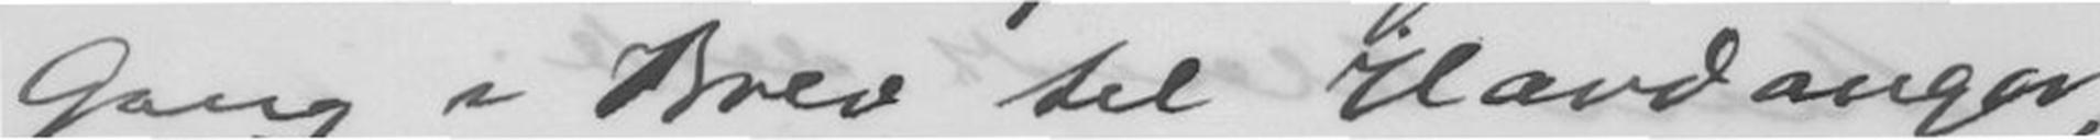Gang i Brev til Hardanger,
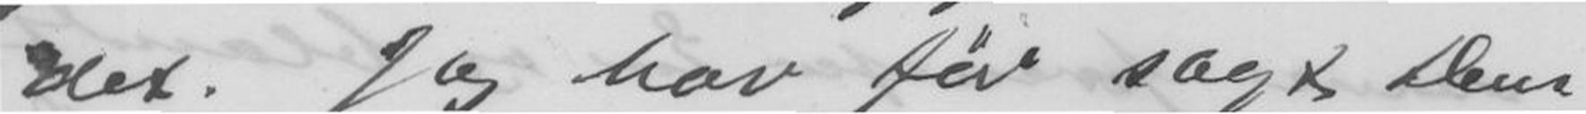det. Jeg har før sagt Dem
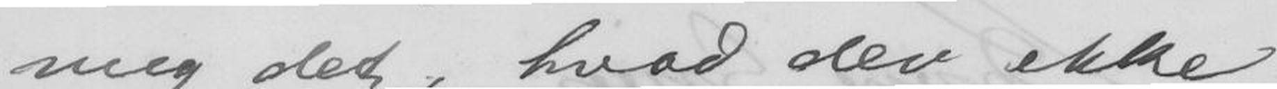mig det, hvad der ikke
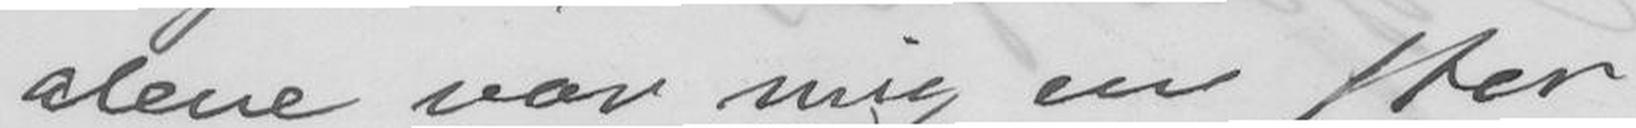alene var mig en stor
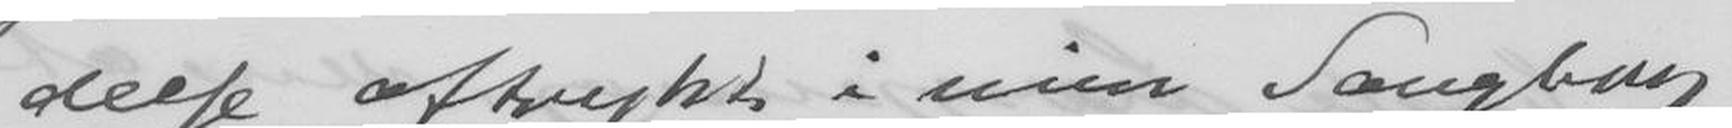delse aftrykket i min Sangbog
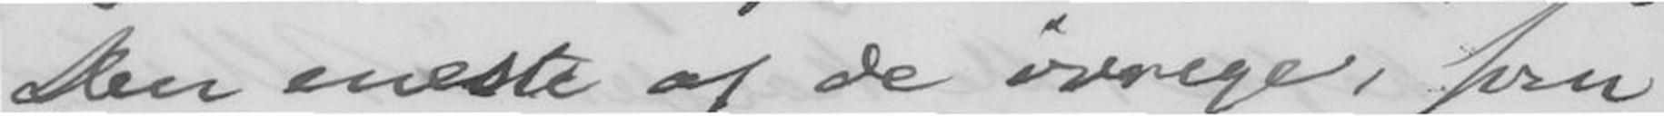Den eneste af de øvrige, som
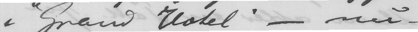i "Grand Hotel" - nu -
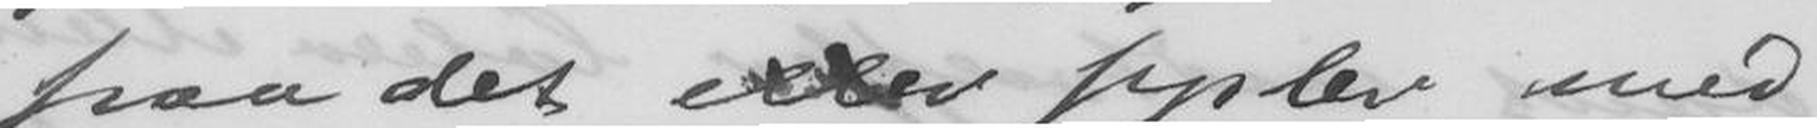paa det eller sysler med
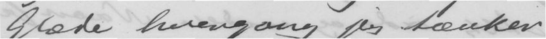Glæde hvergang jeg tænker
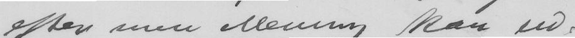efter min Mening kan ud-
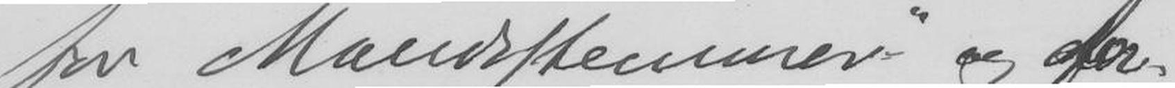for Mandsstemmer" og for
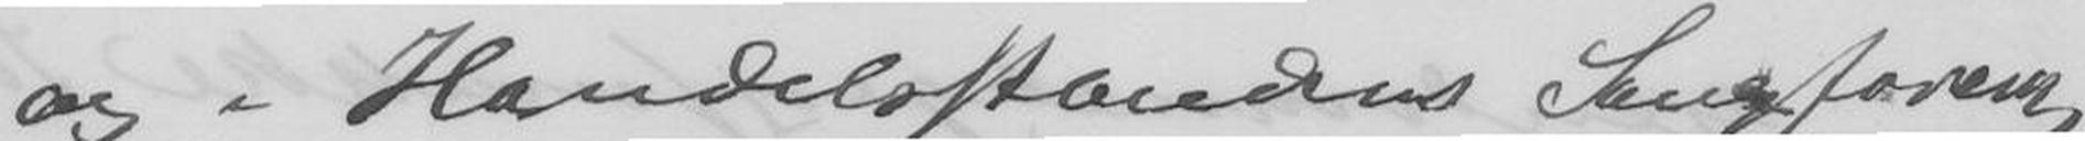og i Handelsstandens Sangforening.
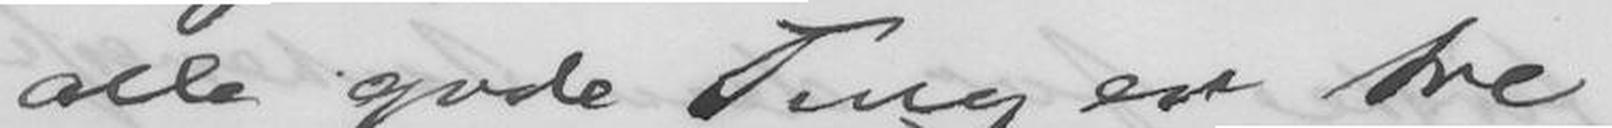alle gode Ting er tre
